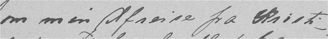om min Afreise fra Kristi-
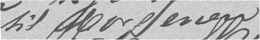til Morgenen
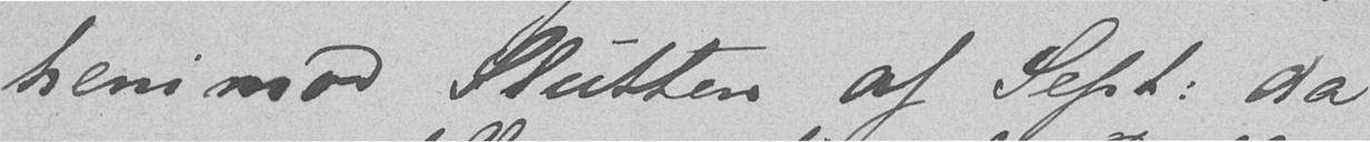henimod Slutten af Sept. da
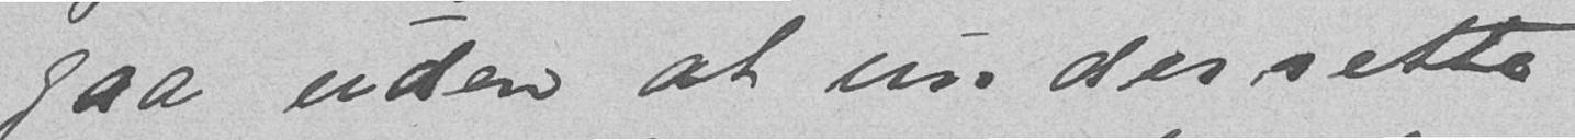gaa uden at underrette
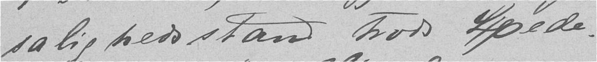salighedsstand trods Hede.
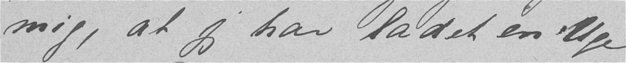mig, at jeg har ladet en Uge
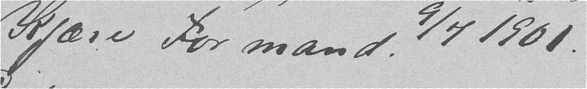Kjære Formand 9/7 1901.
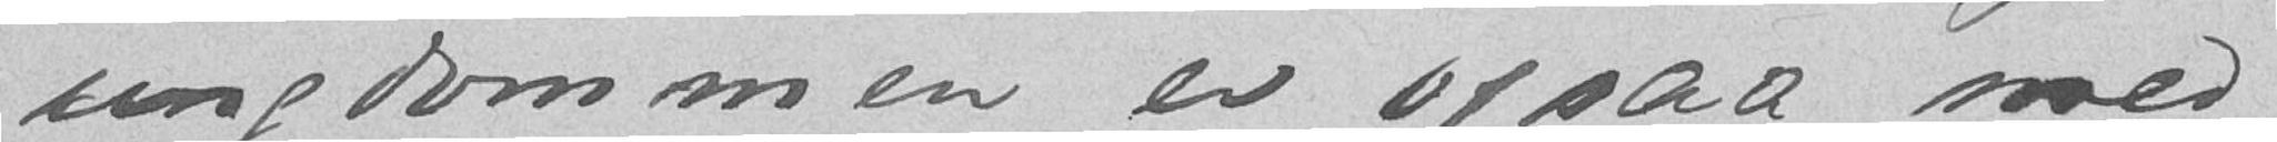ungdommen er ogsaa med
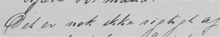Det er nok ikke rigtigt af
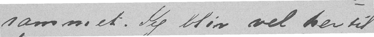rammet. Jeg blir vel her til
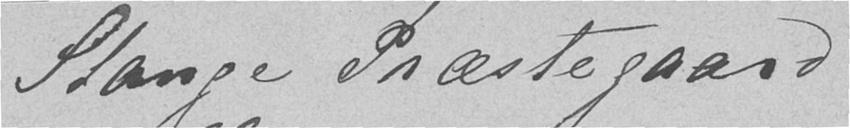Stange Præstegaard
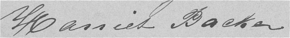Harriet Backer
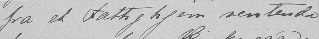fra et Fattighjem ventende
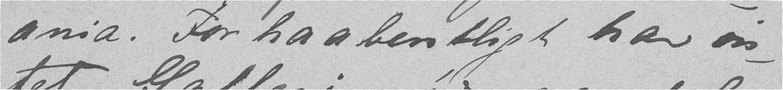ania. Forhaabentligt har in-
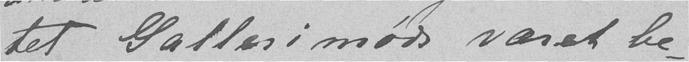tet Gallerimøde været be-
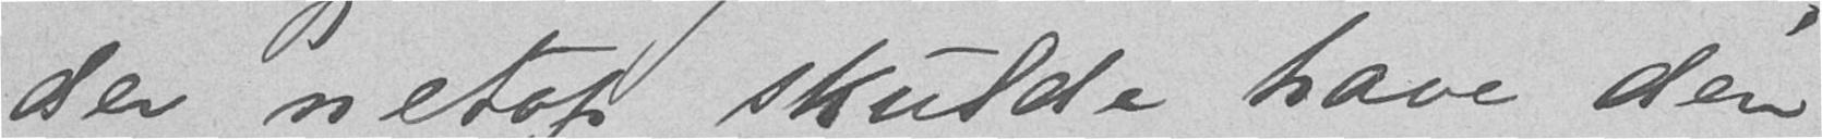der netop skulde have den
